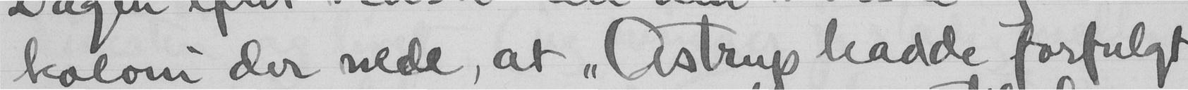koloni der nede, at "Astrup hadde forfulgt
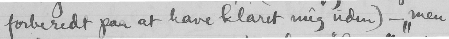forberedt paa at have klaret mig uden) - "men
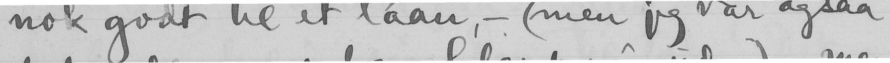nok godt til et laan, - (men jeg var ogsaa
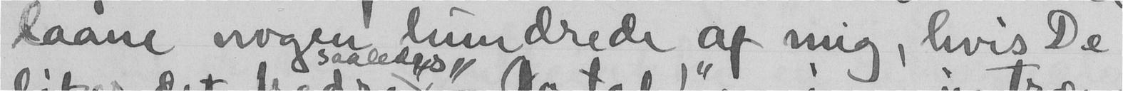laane nogen hundrede af mig, hvis De
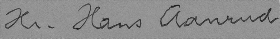Hr. Hans Aanrud
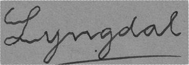Lyngdal
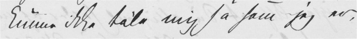kunne ikke tåle mig så som jeg er,
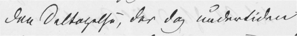den Deltagelse, der dog undertiden
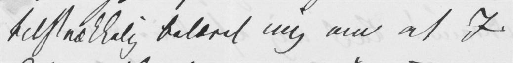tilstrækkelig belæret mig om at I
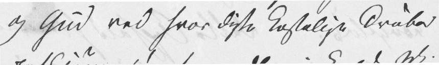og Gud ved hvor disse kostelige Dråber
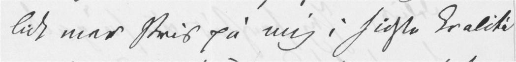lidt mer Pris på mig i sidste Kvaliti
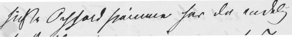sidste Ophold hjemme har da endelig
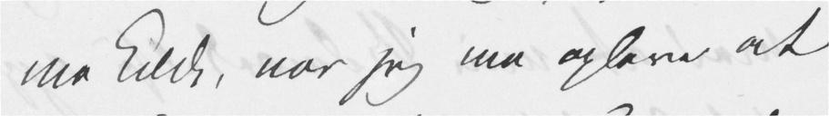me Kilde, nar jeg ma opleve at
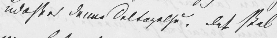udæsker denne Deltagelse. Det skal
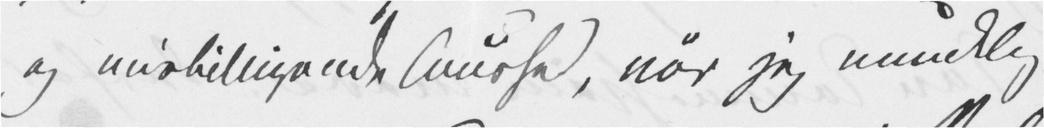og misbilligende Taushed, når jeg endelig
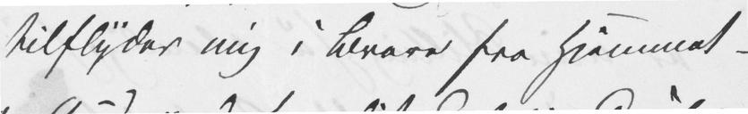tilflyder mig i Breve fra Hjemmet
